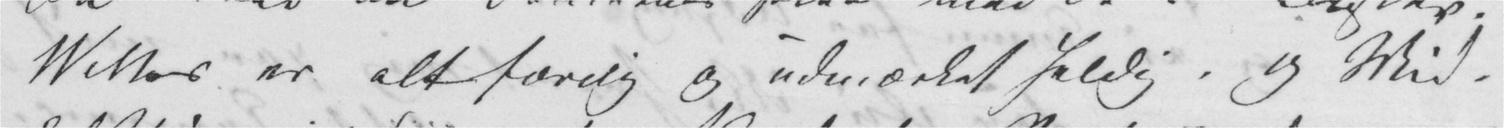Welh.s er alt færdig og udmærket heldig, og
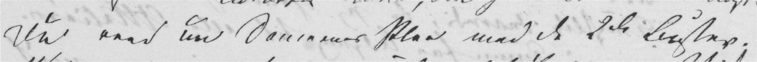Du veed nu Damernes Plan med de tvende Buster.
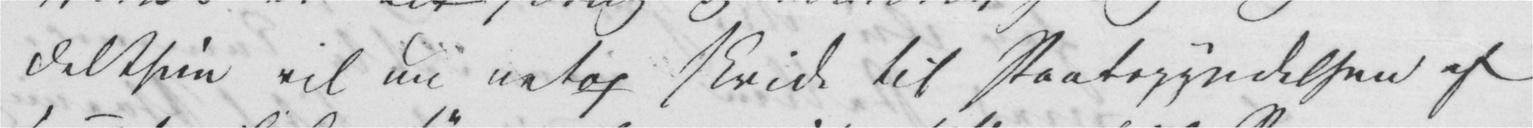Middelthun vil nu netop skride til Paabegyndelsen af
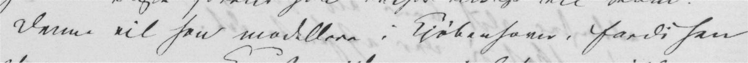Denne vil han modellere i Kjøbenhavn, fordi han
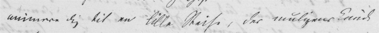animere Dig til en lille Reise, der muligens kunde
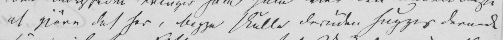at gjøre det her, begge skulle desuden hugges dernede
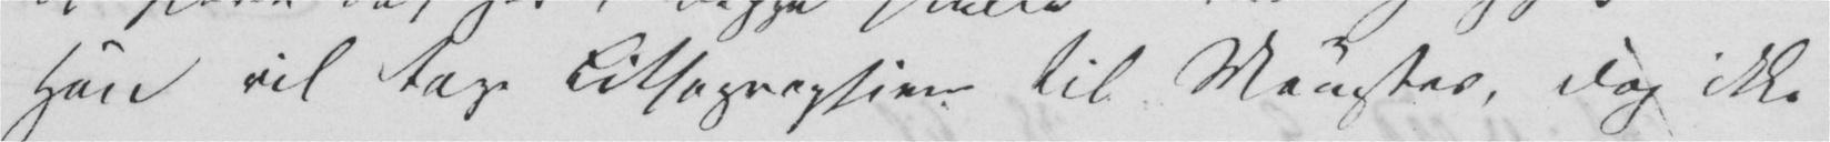Han vil tage Lithographien til Mønster, dog ikke
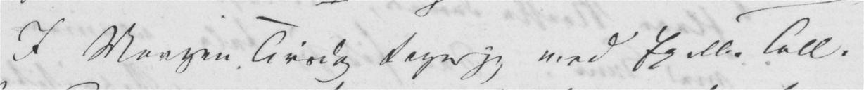I Morgen Tirsdag tager jeg med Ex eller Toll.
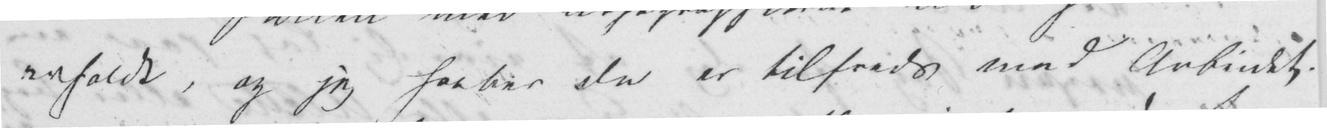erholdt, og jeg haaber Du er tilfreds med Arbeidet.
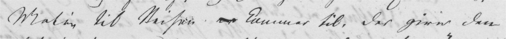Motiv til Reisen er kommer til, der giver den
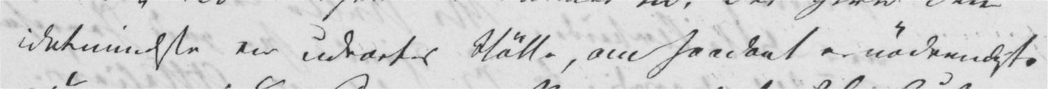idetmindste en udvortes Støtte, om saadant er nødvendigt.
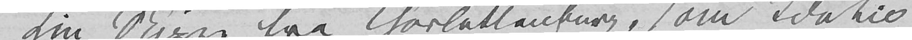Hin Skizze fra Charlottenburg, som Ida Lie
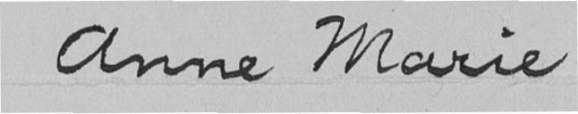Anne Marie
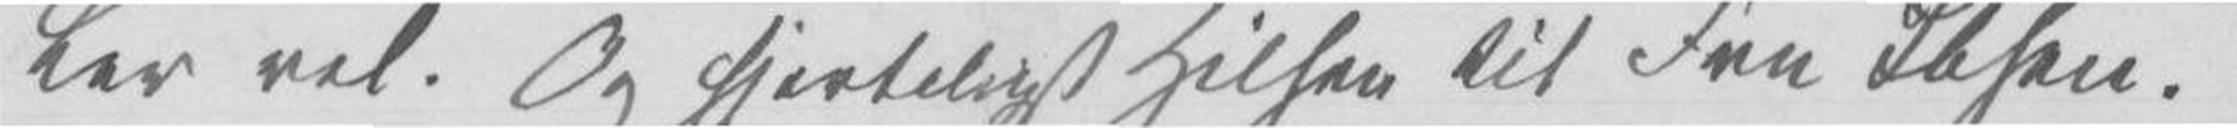Lev vel. Og hjerteligst Hilsen til Fru Ibsen.
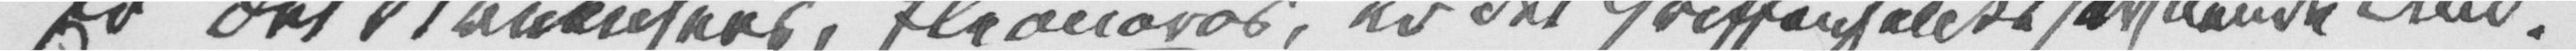Er det Struensees, Eleonoras, er det Griffenfeldts hævnende Ånd.
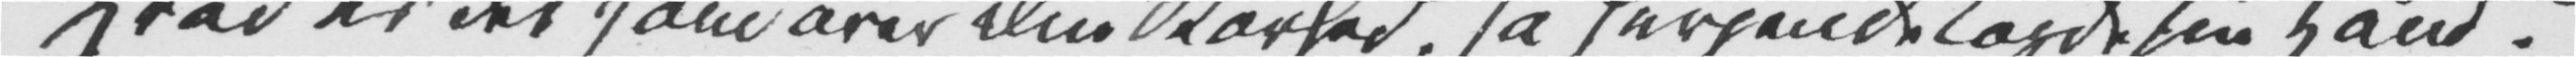Hvad er det som over din Storhed, så herjende lagde sin Hånd?
